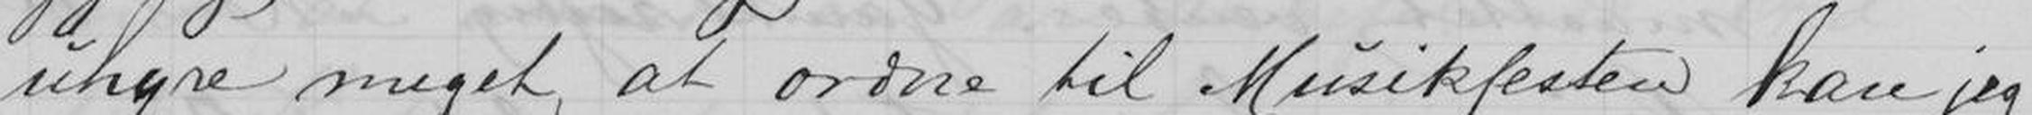uhyre meget at ordne til Musikfesten kan jeg
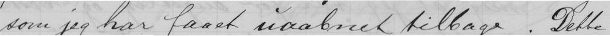som jeg har faaet uaabnet tilbage. Dette
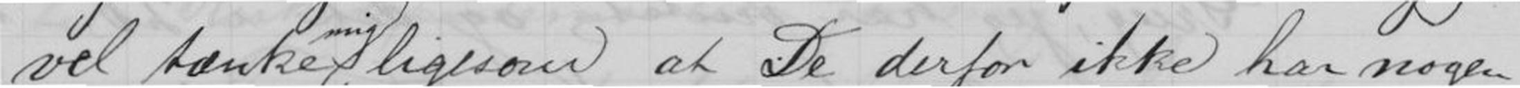vel tænke, ligesom at De derfor ikke har nogen
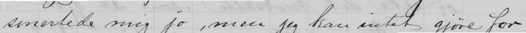smertede mig jo, men jeg kan intet gjøre for
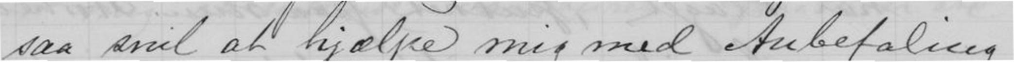saa snil at hjælpe mig med Anbefaling
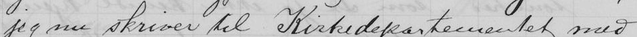jeg nu skriver til Kirkedepartementet med
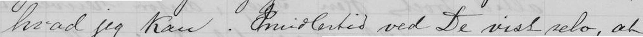hvad jeg kan. Imidlertid ved De vist selv, at
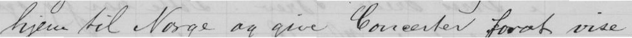hjem til Norge og give Concerter forat vise,
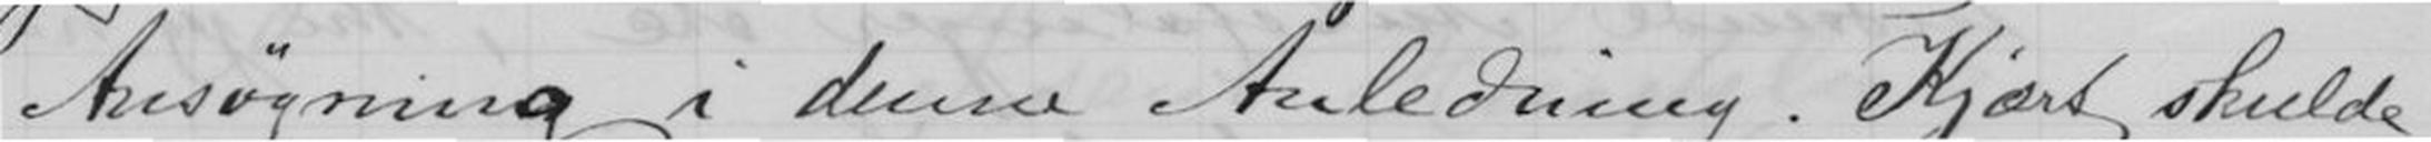Ansøgning i denne Anledning. Kjært skulde
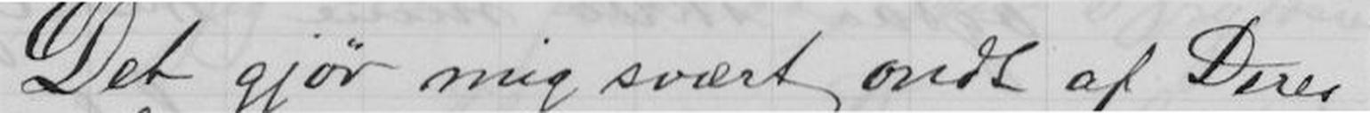Det gjør mig svært ondt af Deres
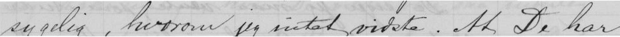sygelig, hvorom jeg intet vidste. At De har
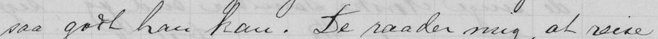saa godt han kan. De raader mig, at reise
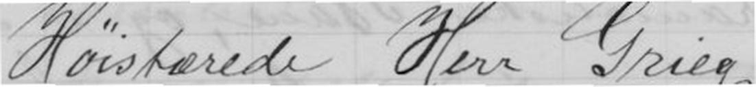Høistærede Herr Grieg!
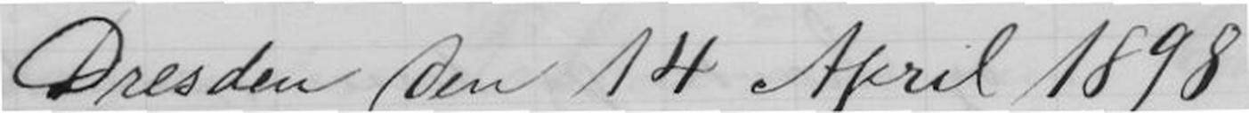Dresden den 14 April 1898
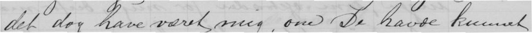det dog have været mig, om De havde kunnet
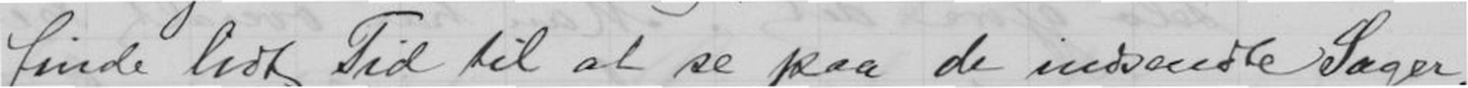finde lidt Tid at se paa de indsendte Sager,
